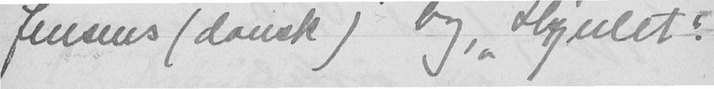Jensens (dansk) bog, "Hjertet".
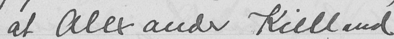at Alexander Kielland
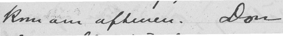kom om aftenen. Don
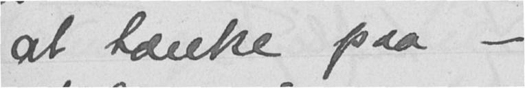at tænke paa -
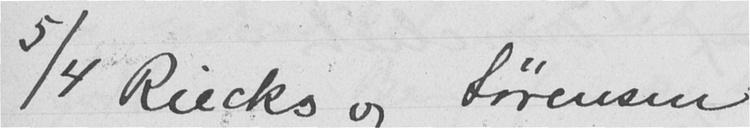5/4 Riecks og Sørensen
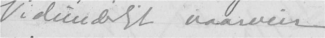Vidunderligt vaarveir.
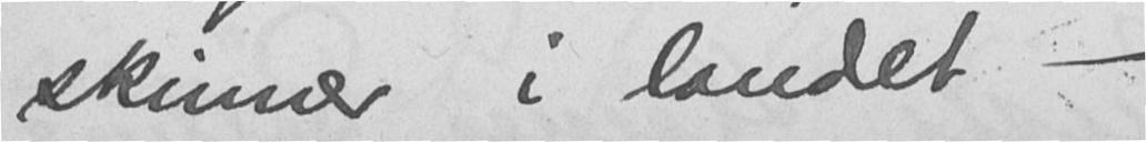skinner i landet -
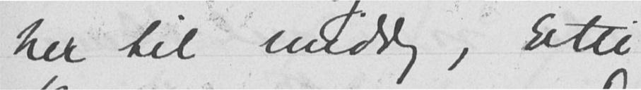her til middag, Etti
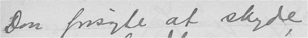Don forsøgte at skyde
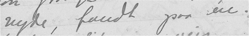rugde, fandt ogsaa én.
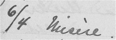6/4 Misère.
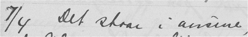7/4 Det staar i avisene,
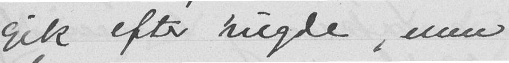gik efter rugde, men
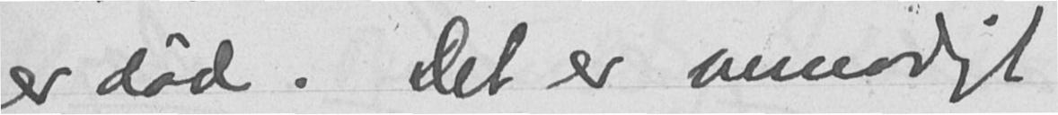er død. Det er vemodigt
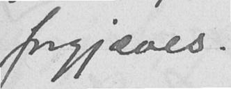forgjæves.
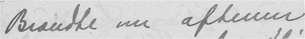Brændte om aftenen
